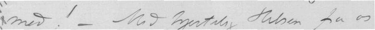med! - Med hjertelig Hilsen fra os
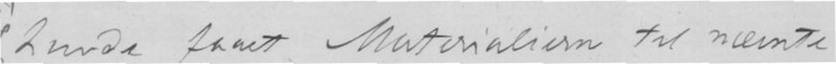havde faaet Materialierne til nævnte
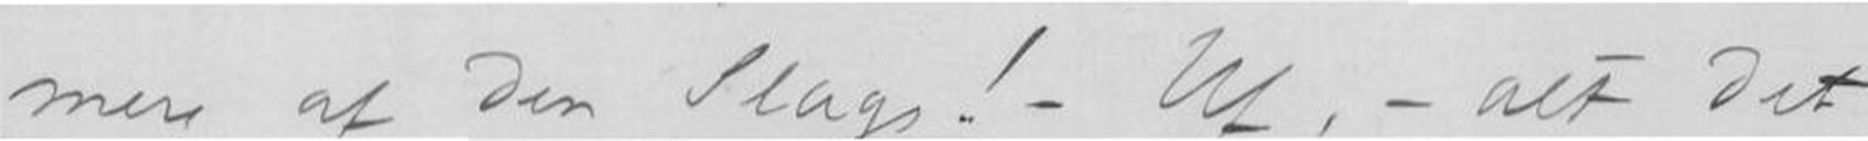mere af den Slags! - Uf, - alt det
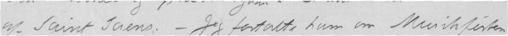af Saint Saens. - Jeg fortalte ham om Musikfesten
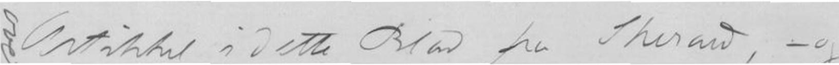Artikkel i dette Blad fra Sherard, - og
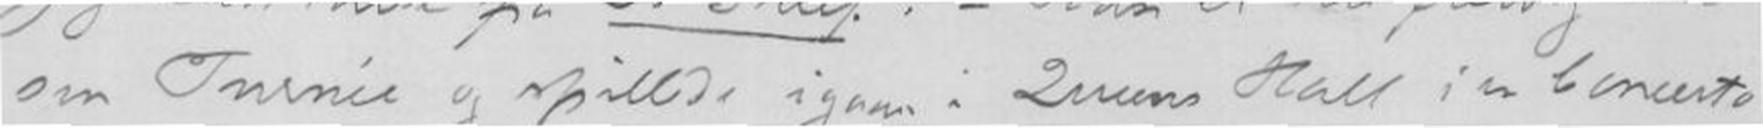sin Turnie og spillede igaar i Queens Hall i en Concerto
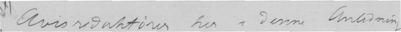Avisredaktører her i denne Anledning,
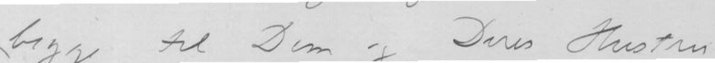begge til Dem og Deres Hustru
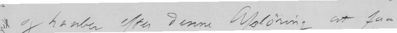og haaber efter denne Afsløring at faa
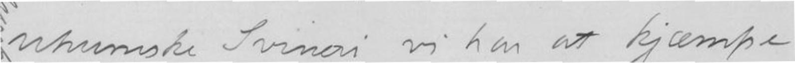uhumske Svineri vi har at kjæmpe
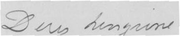Deres hengivne
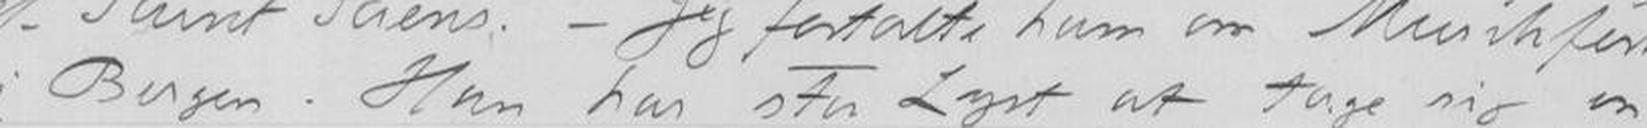i Bergen. Han har stor Lyst at tage sig en
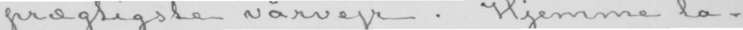prægtigste vårvejr. Hjemme la-
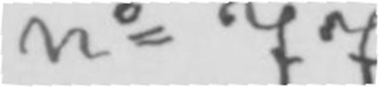no 77.
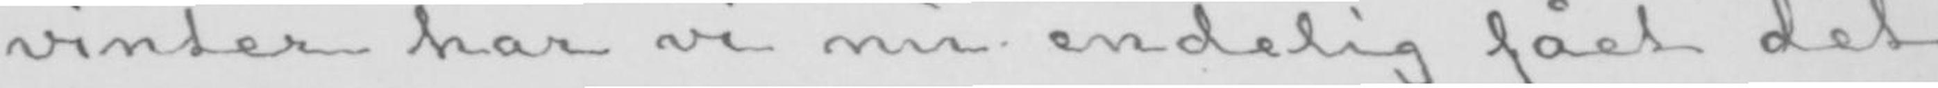vinter har vi nu endelig fået det
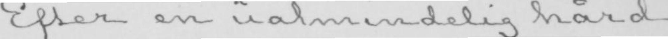Efter en ualmindelig hård
